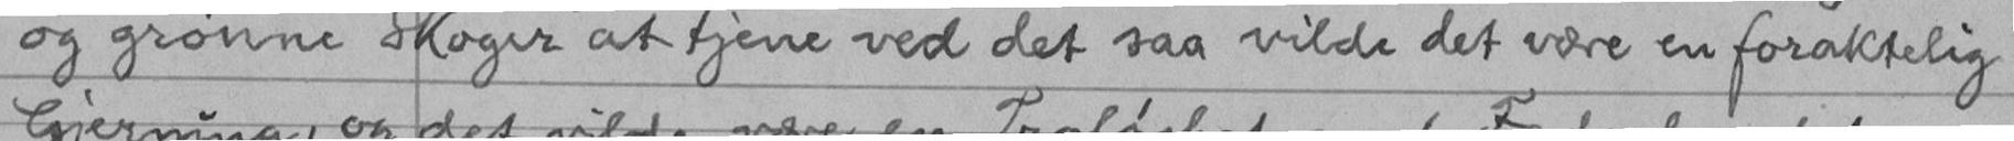og grønne Skoger at tjene ved det saa vilde det være en foraktelig
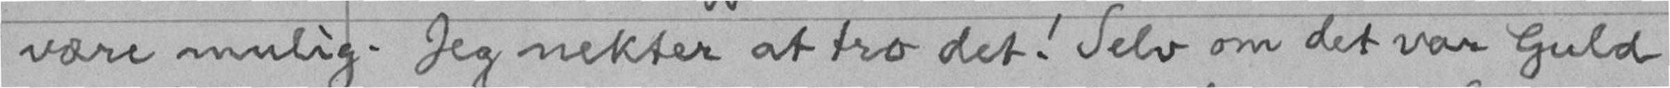være mulig. Jeg nekter at tro det! Selv om det var Guld
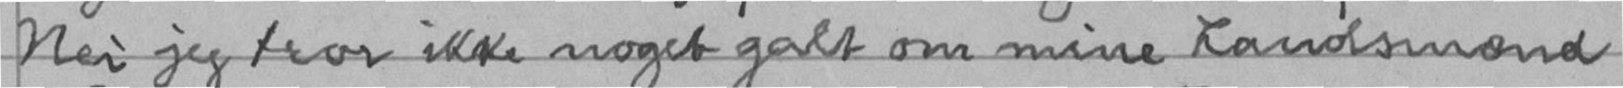Nei jeg tror ikke noget galt om mine Landsmænd
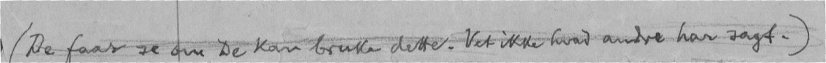(De faar se om De kan bruke dette. Vet ikke hvad andre har sagt.)
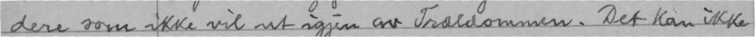dere som ikke vil ut igjen av Trældommen. Det kan ikke
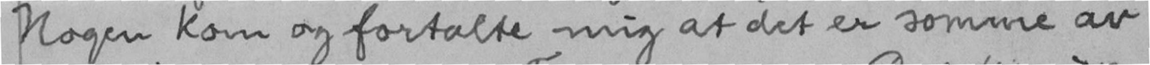Nogen kom og fortalte mig at det er somme av
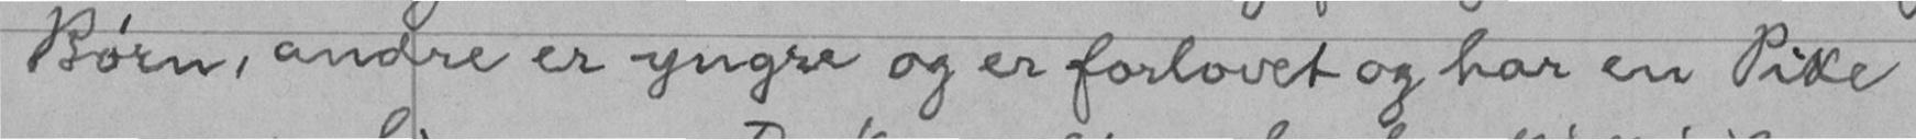Børn, andre er yngre og er forlovet og har en Pike
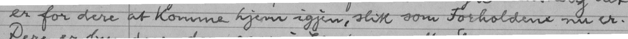er for dere at komme hjem igjen, slik som Forholdene nu er.
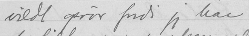vildt oprør fordi jeg har
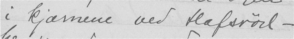i kjærnene ved Hafsrød -
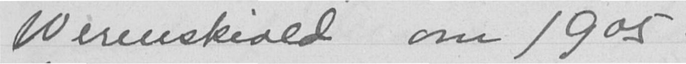Werenskiold om 1905.
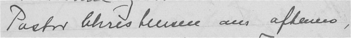Pastor Christensen om aftenen,
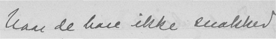Naar de bare ikke snakked
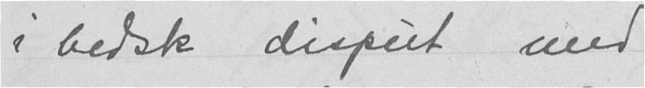i bedsk disput med
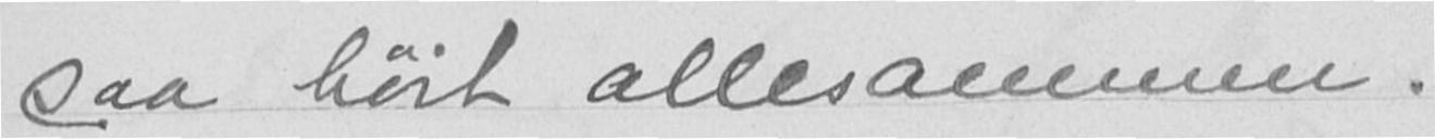saa høit allesammen.
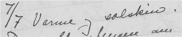7/7 Varme og solskin.
